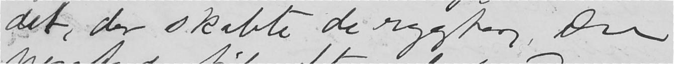det, der skabte de rygter, Du
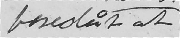foreslåt at
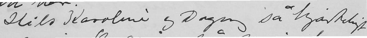Hils Karoline og Dagny så hjærteligt
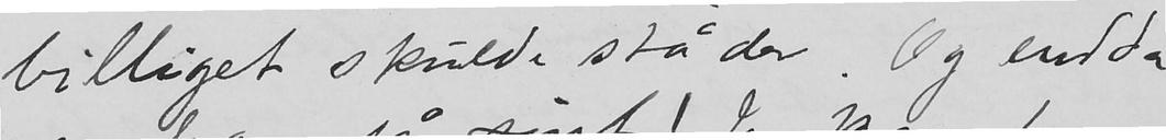billiget skulde stå der. Og endda
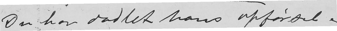Du har dadlet hans opførsel og
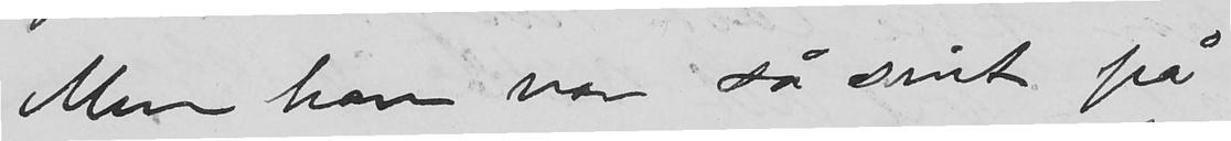Men han var så sint på
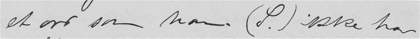et ord som han (S.) ikke har
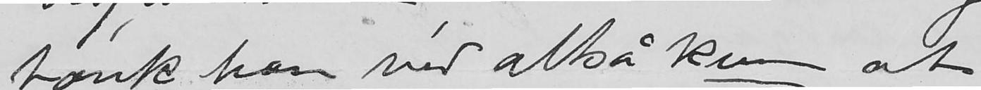tænk han véd altså kun at
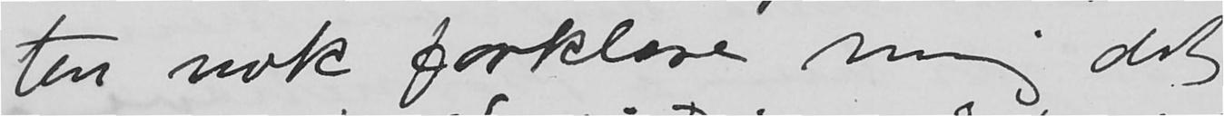ten nok forklare mig det,
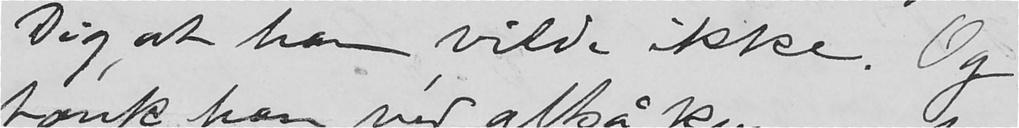Dig at han vilde ikke. Og
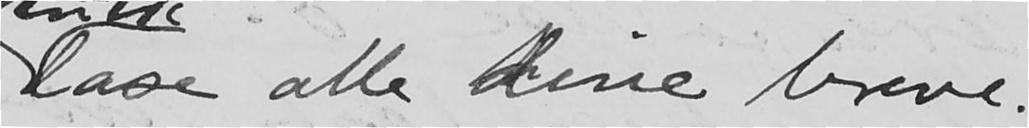læse alle dine breve.
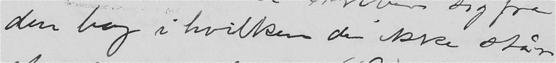den bog i hvilken der ikke står
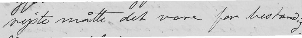rejste måtte det være for bestandig.
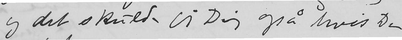og det skulde jeg Dig også hvis Du
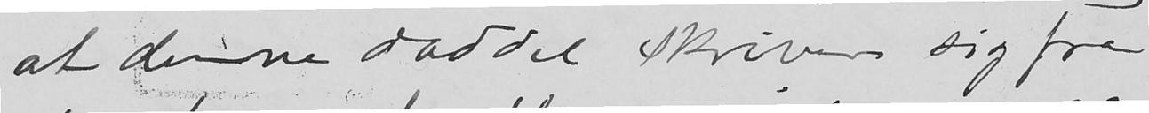at denne daddel skriver sig fra
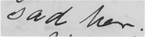sad her.
